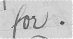for.
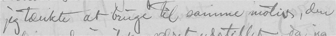jeg tænkte at bruge til samme motiv, - den
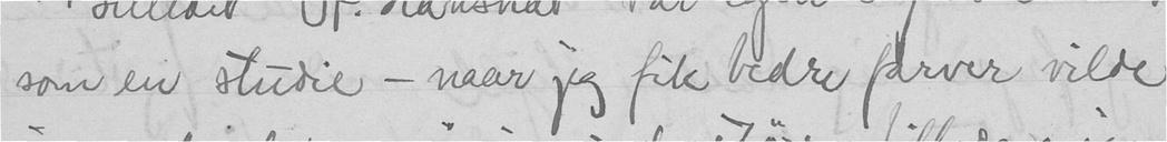som en studie - naar jeg fik bedre farver vilde
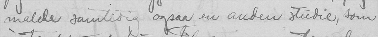malde samtidig ogsaa en anden studie, som
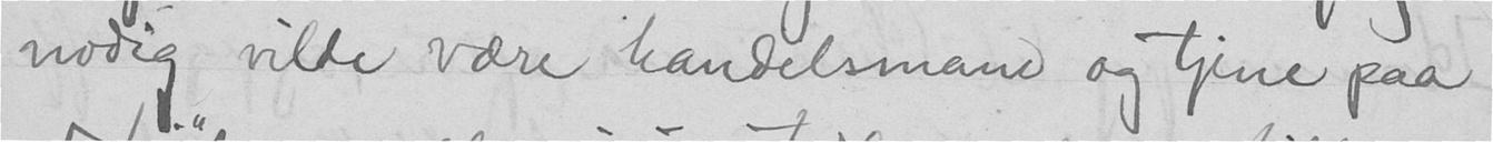nødig vilde være handelsmand og tjene paa
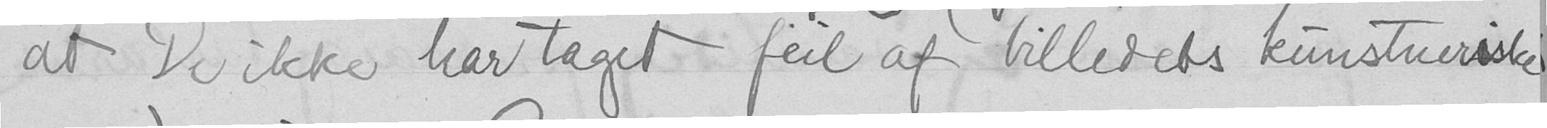at De ikke har taget feil af billedets kunstneriske
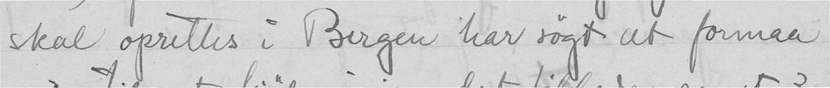skal oprettes i Bergen har søgt at formaa
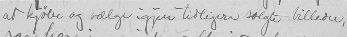at kjøbe og sælge igjen tidligere solgte billeder,
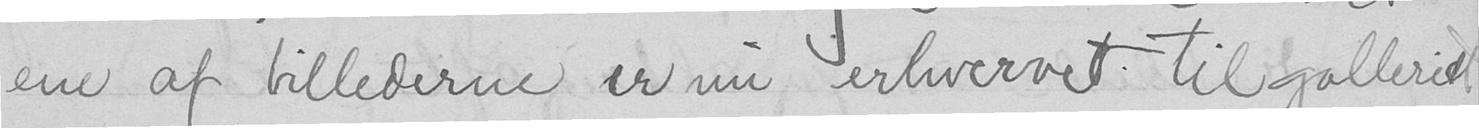ene af billederne er nu erhvervet til galleriet
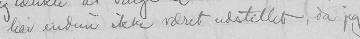har endnu ikke været udstillet, da jeg
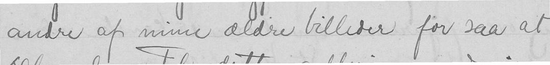andre af mine ældre billeder for saa at
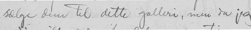sælge dem til dette galleri, men da jeg
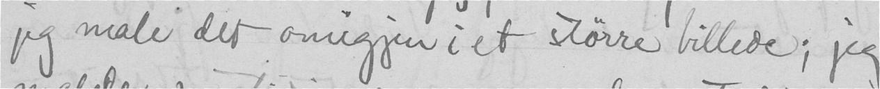jeg male det omigjen i et større billede, jeg
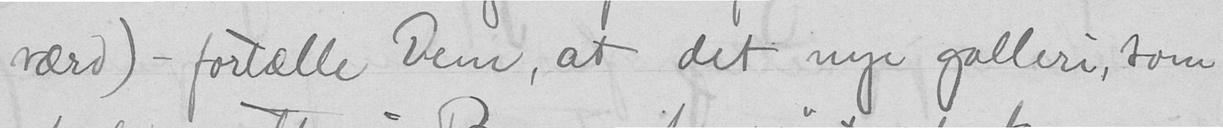værd) - fortælle Dem, at det nye galleri, som
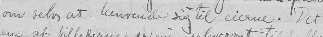om selv at henvende sig til eierne. - Det
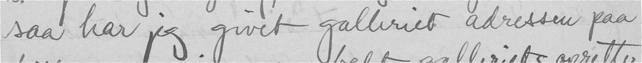saa har jeg givet galleriet adressen paa
